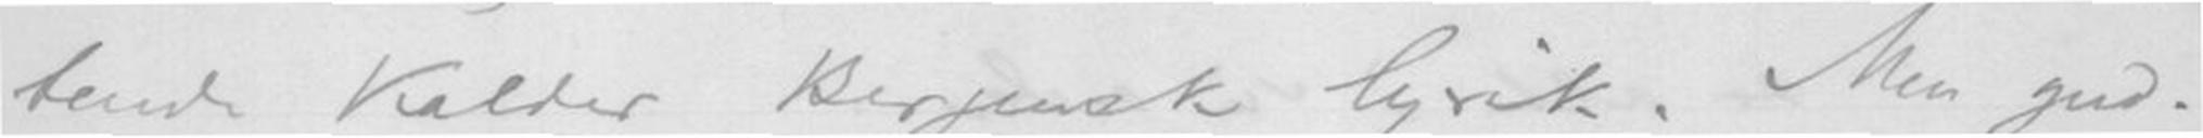tende kalder Bergensk Lyrik. Men gud-
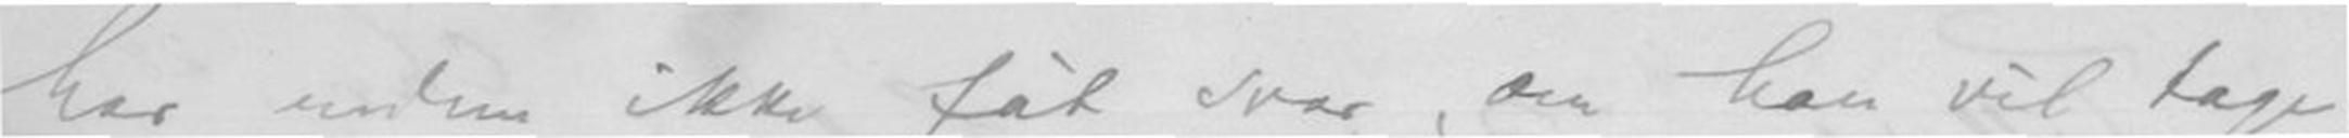har endnu ikke fåt svar, om han vil tage
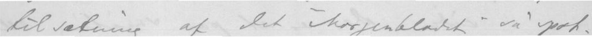tilsætning af det "Morgenbladet" så spot-
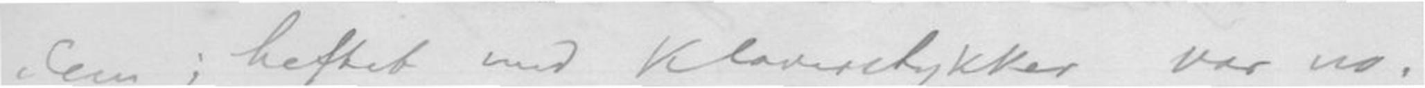dem; heftet med Klaverstykker var no-
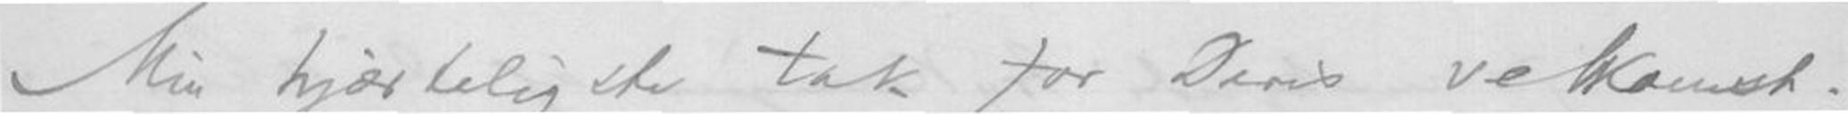Min hjærteligste tak for Deres velkomst
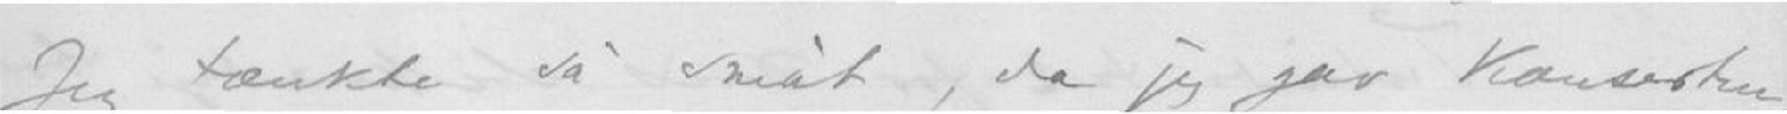Jeg tænkte så småt, da jeg gav Konserten,
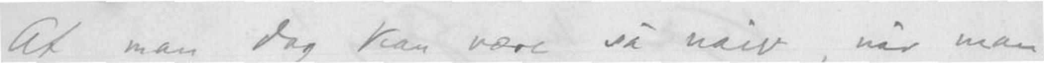At man dog kan være så naiv, når man
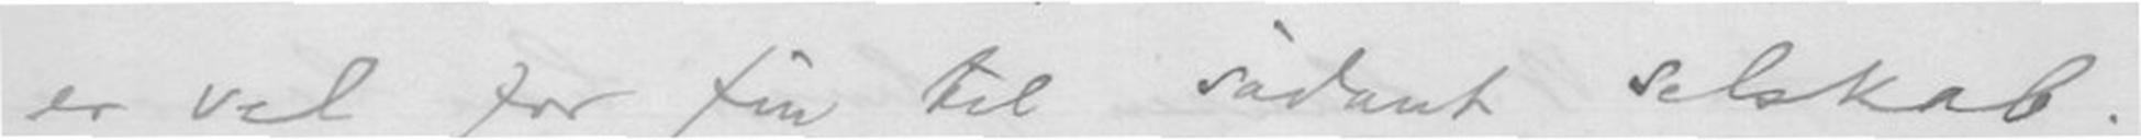er vel for fin til sådant selskab.
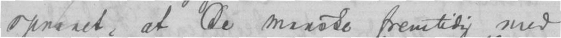opnaaet, at De maaske fremtidig med
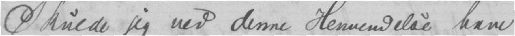Skulde jeg ved denne henvendelse have
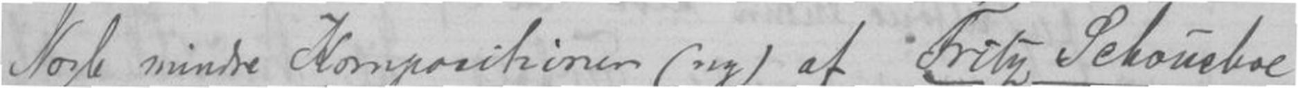Nogle mindre Kompositioner (avg) af Fritz Schouskoe??
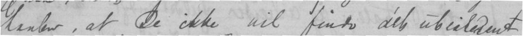haaber, at de ikke vil finde det ,
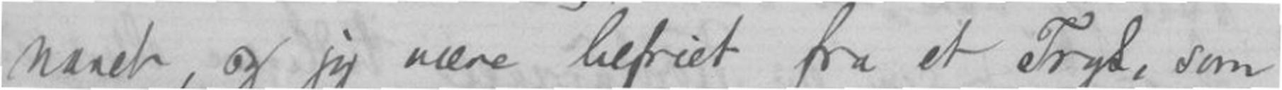naaet, og jeg være befriet fra et Fryd, som
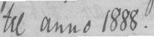til anno 1888.
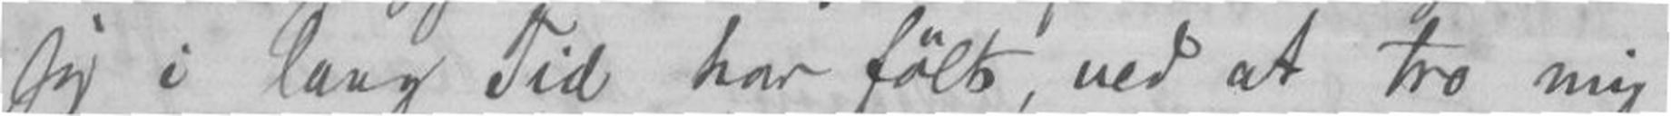jeg i lang Tid har følt, ved at tro mig
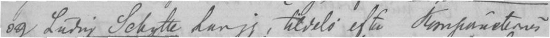og Ludivg Schytte , tilvels efter Konpositionen
